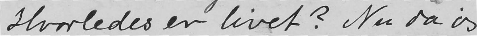Hvorledes er livet? Nu da jeg
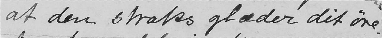at den straks glæder dit øre.
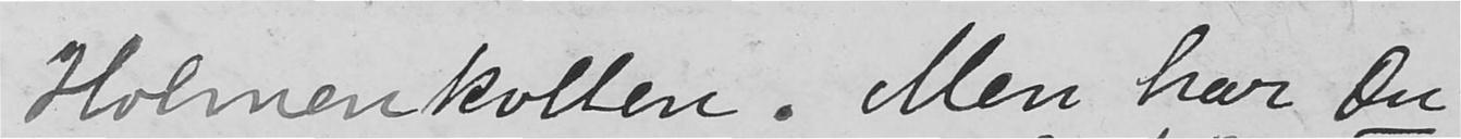Holmenkollen. Men har Du
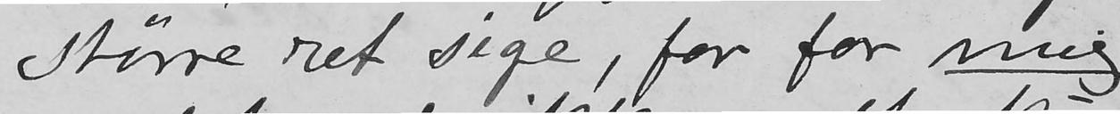større ret sige, for for mig
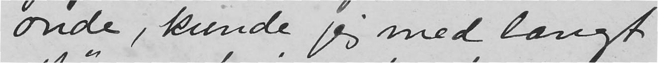onde, kunde jeg med langt
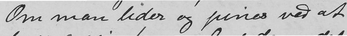Om man lider og pines ved at
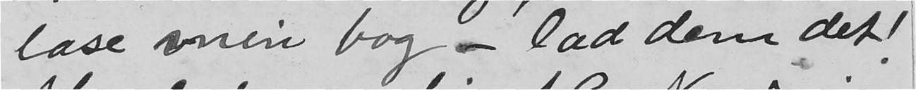læse min bog - lad dem det!
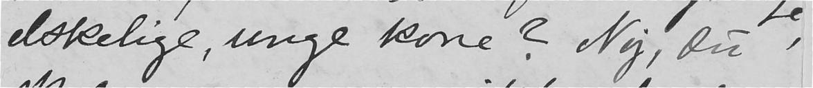elskelige, unge kone? Nej, Du,
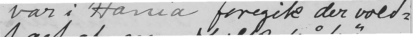var i Xania foregik der vold-
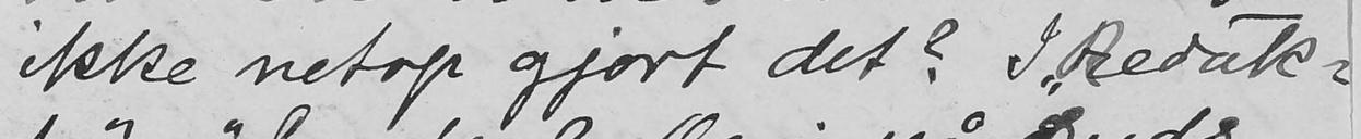ikke netop gjort det? I "Redak-
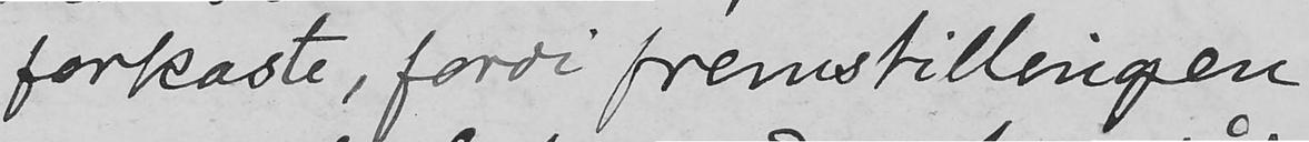forkaste, fordi fremstillengen
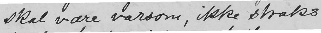skal være varsom, ikke straks
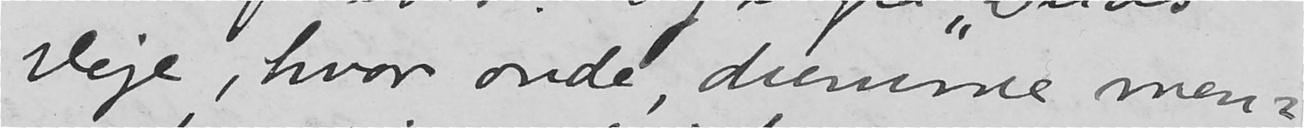Veje, hvor onde dumme men-
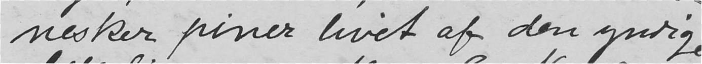nesker piner livet af den yndige
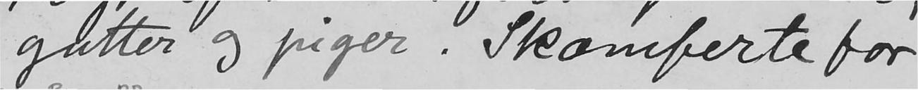gutter og piger. Skamferte for
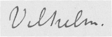Vilhelm.
